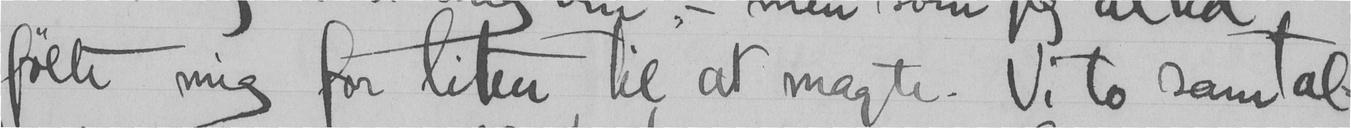følte mig for liten til at magte. Vi to samtal-
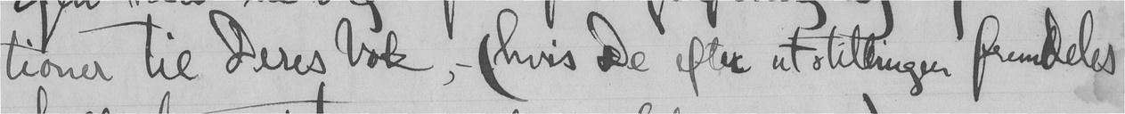tioner til Deres bok - (hvis de efter utstillingen fremdels
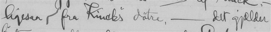ligesaa,- fra Kincks døtre, - det gjælder
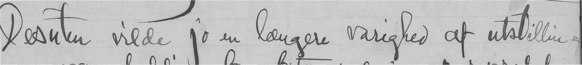Desuten vilde jo en længere varighed af utstilin-
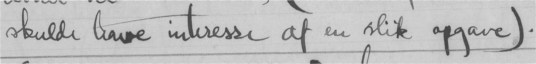skulde have interesse af en slik opgave).
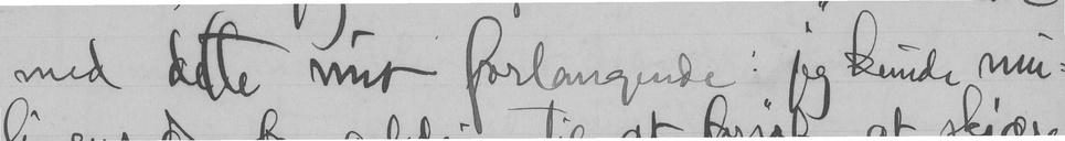med dette mit forlangende: jeg kunde mu-
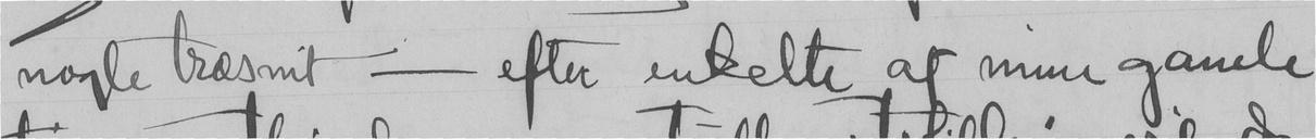nogle træsnit - efter enkelte af mine gamle
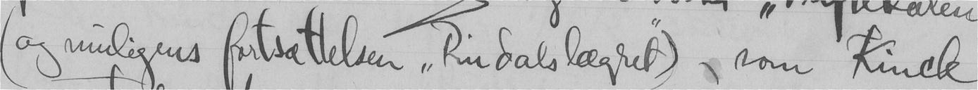(og muligens fortsættelsen "Rindalslægret"), som Kinck
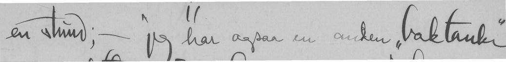en stund; - jeg har ogsaa en anden "baktanke"
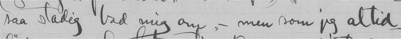saa stadig bad mig om, - men som jeg altid
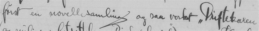først en novellesamling og saa verket "Driftekaren"
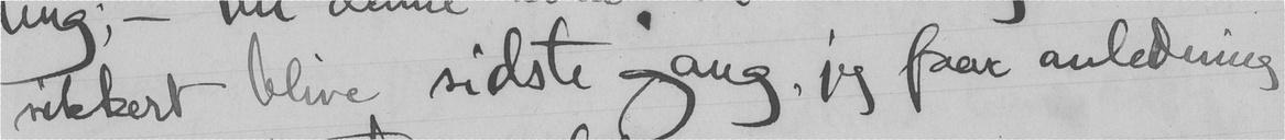sikkert blive sidste gang, jeg faar anledning
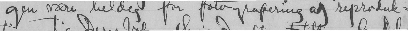gen være heldig for fotografernig og reproduk-
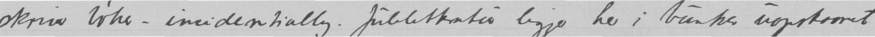skriver bøker – incidentially. Juleletkratur ligger her i bunker uopskaaret
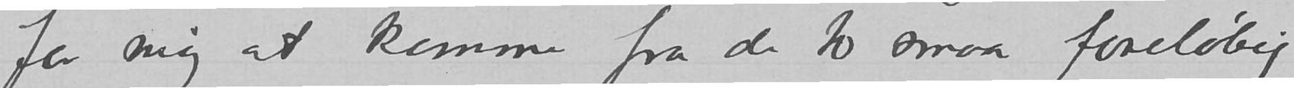for mig at komme fra de to smaa foreløbig.
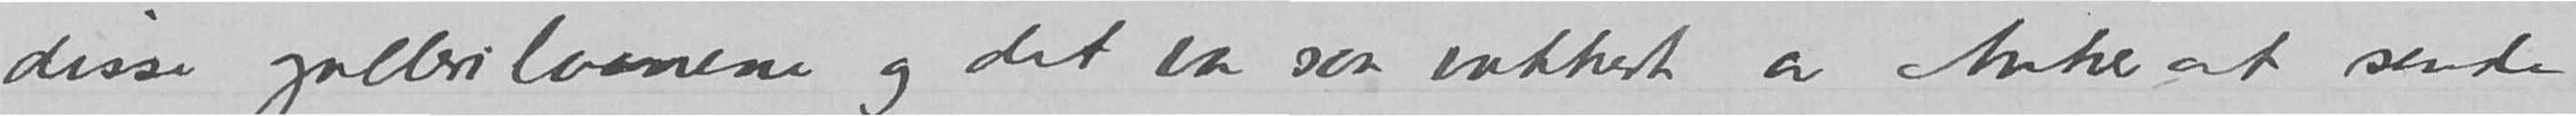disse gallerilaanene og det var saa vakkert av Anker at sende
